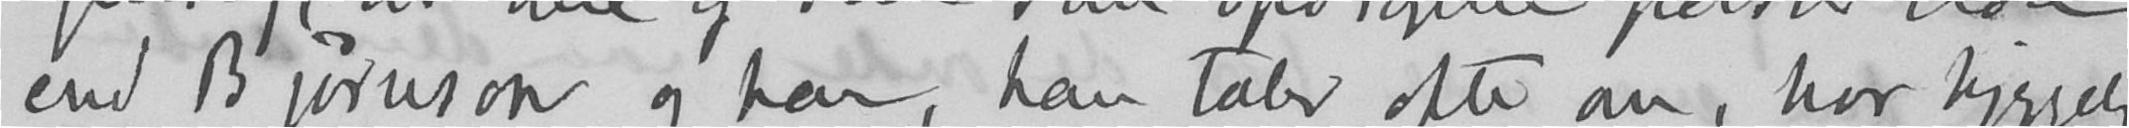end Bjørnson og han, han taler ofte om, hvor hyggelig
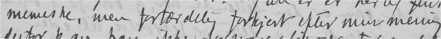menneske, men forfærdelig forkjert efter min mening,
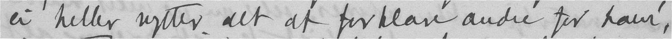ei heller nytter det at forklare andre for ham,
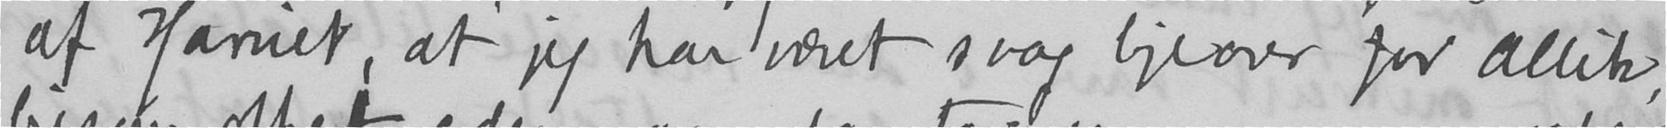af Harriet, at jeg har været svag ligeover for Allik,
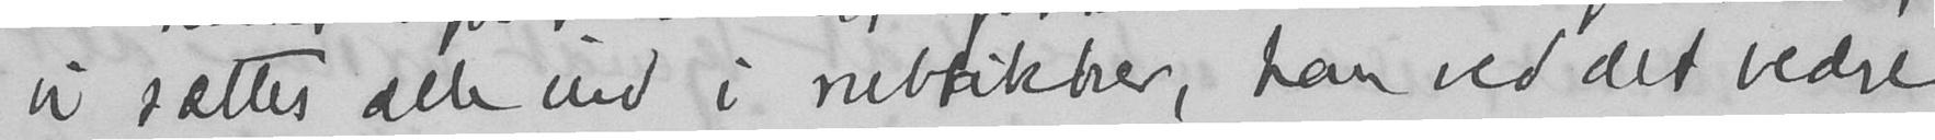vi sættes alle ind i rubrikker, han ved det bedre
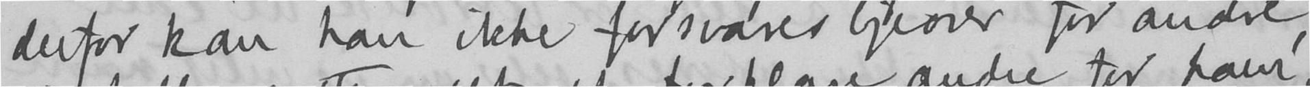derfor kan han ikke forsvares ligeover for andre,
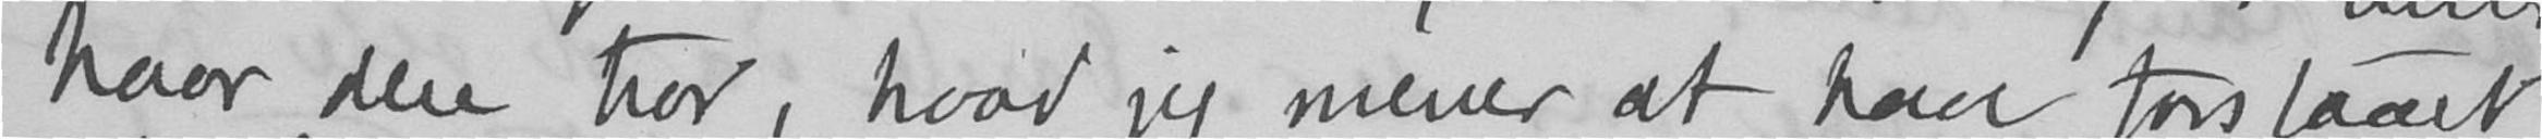Naar dere tror, hvad jeg mener at have forstaaet
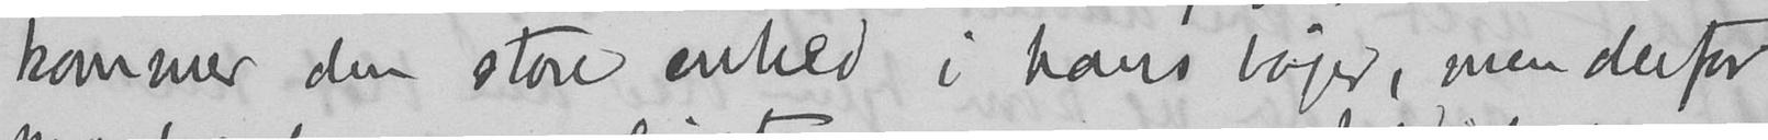kommer den store enhed i hans bøger, men derfor
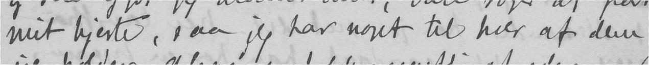mit hjerte, saa jeg har noget til hver af dem
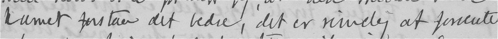kunnet forstaa det bedre, det er rimelig af forvente
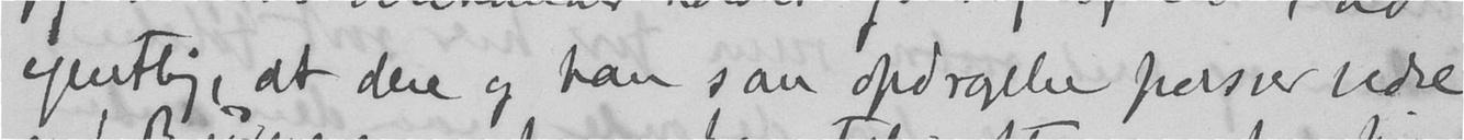egentlig, at dere og han sin opdragelse passer bedre
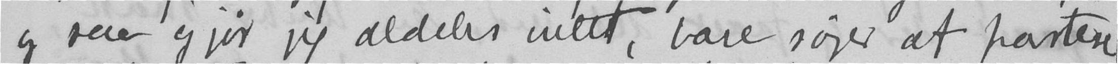og saa gjør jeg aldeles intet, bare søger at partere
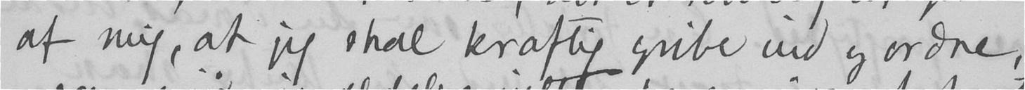af mig, at jeg skal kraftig gribe ind og ordne,
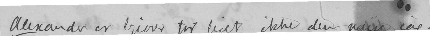Alexander er ligeover for livet ikke den naive iag-
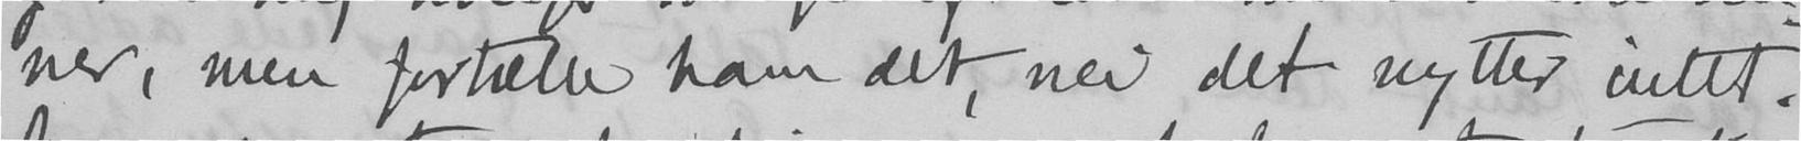ner, men fortælle ham det, nei det nytter intet.
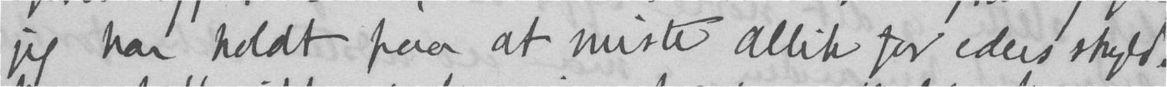jeg har holdt paa at miste Allik for eders skyld.
